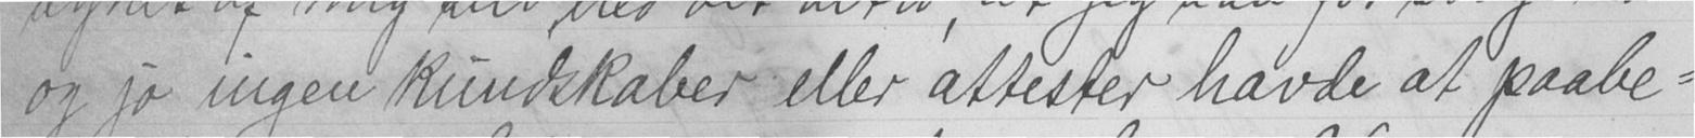og jo ingen kundskaber eller attester havde at paabe-
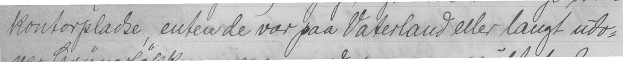kontorpladse, enten de var paa Vaterland eller langt udo-
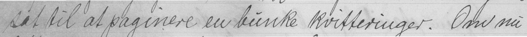sat til at paginere en bunke kvitteringer. Om nu
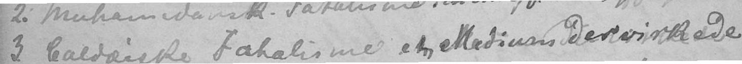3. Caldæiske Fatalisme et Medium der virkede
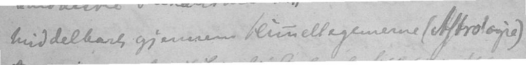middelbart gjennem Himmellegemerne (Astrologie)
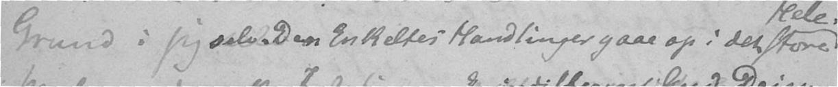Grund i sig selv. Den Enkeltes Handlinger gaar op i det store
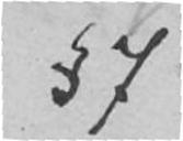§ 7
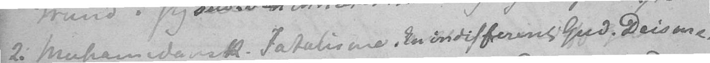2. Muhamedansk. Fatalisme. En indifferent Gud. Deisme.
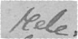Hele.
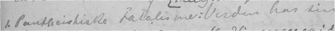1. Pantheistiske Fatalisme: Verden har sin
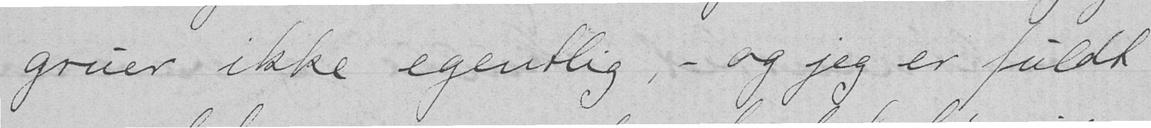gruer ikke egentlig, - og jeg er fuldt
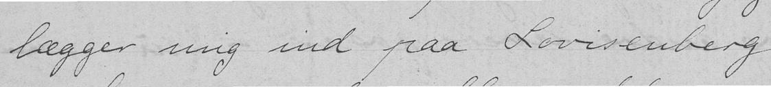lægger mig ind paa Lovisenberg
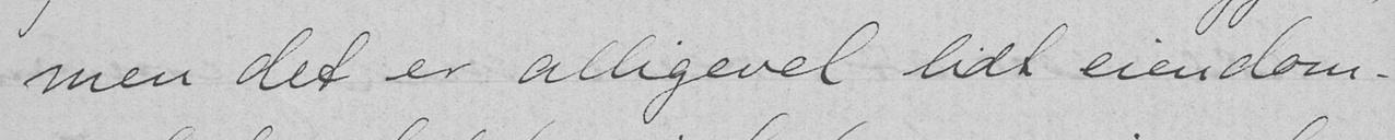men det er alligevel lidt eiendom-
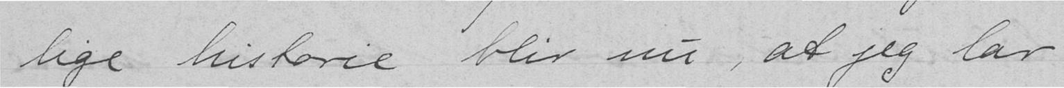lige historie blir nu, at jeg lar
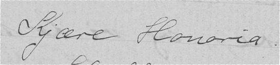Kjære Honoria!
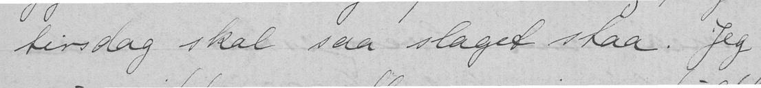tirsdag skal saa slaget staa. Jeg
